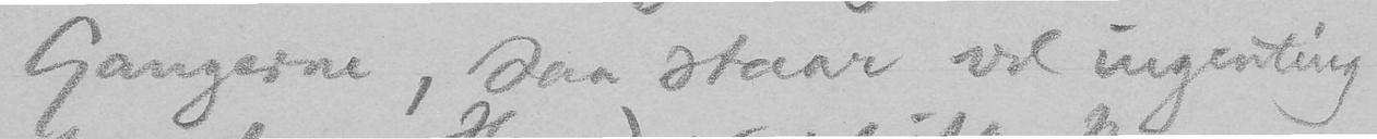Gangerne, saa staar vel ingenting
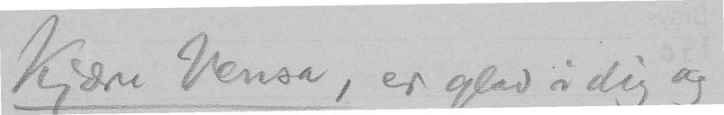Kjære Vensa, er glad i dig og
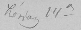Lørdag 14de
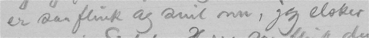er saa flink og snil nu, jeg elsker
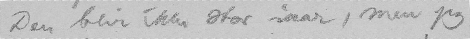Den blir ikke stor iaar, men jeg
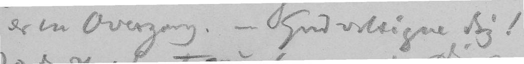er en Overgang. - Gud velsigne dig!
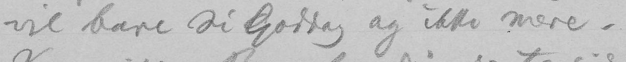vil bare si Goddag og ikke mere.
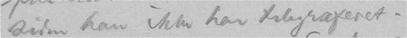siden han ikke har telegraferet.
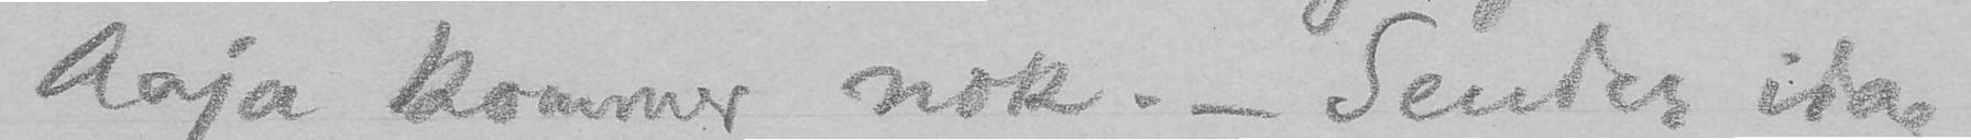Aaja kommer nok. - Sender idag
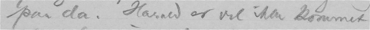paa da. Harald er vel ikke kommet
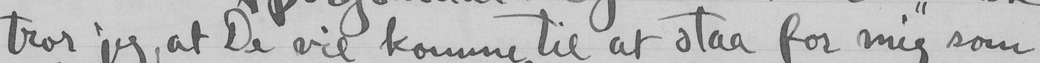tror jeg, at De vel komme til at staa for mig som
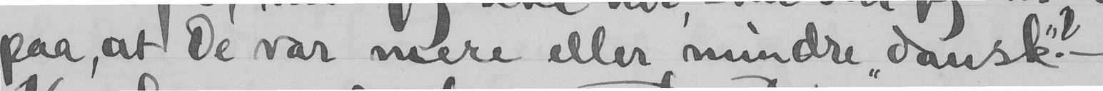paa, at De var mere eller mindre "dansk"? -
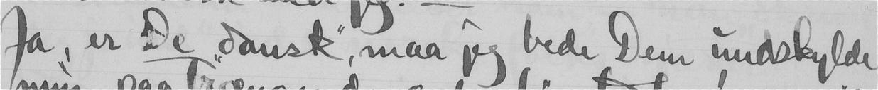Ja, er De "dansk", maa jeg bede Dem undskylde
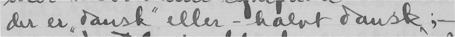der er "dansk" eller - halvt dansk; -
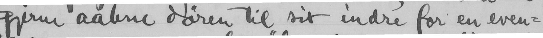gjerne aabne døren til sit indre for en even-
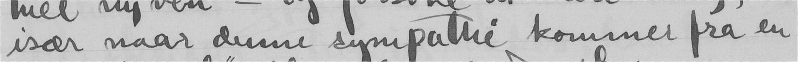især naar denne sympathi kommer fra en
